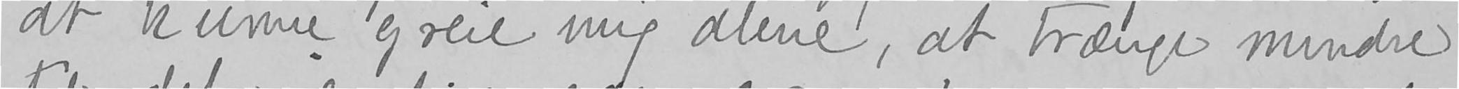at kunne greie mig alene, at trænge mindre
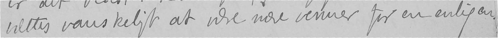vættes vanskeligt at være nære venner for en enlig en.
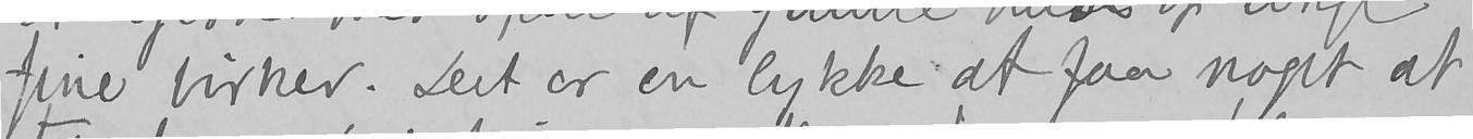fine birker. Det er en lykke at faa noget at
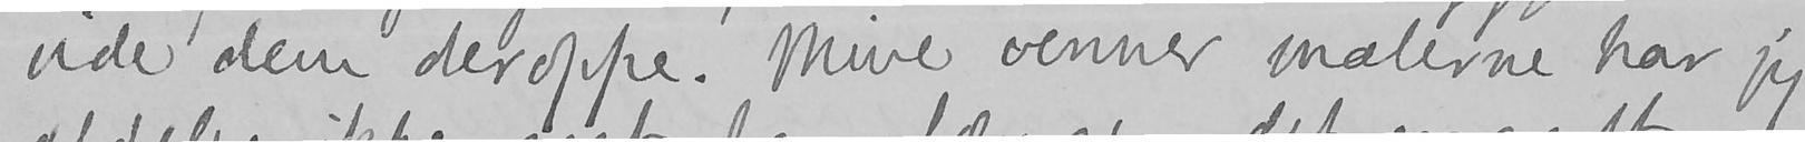vide dem deroppe. Mine venner malerne har jeg
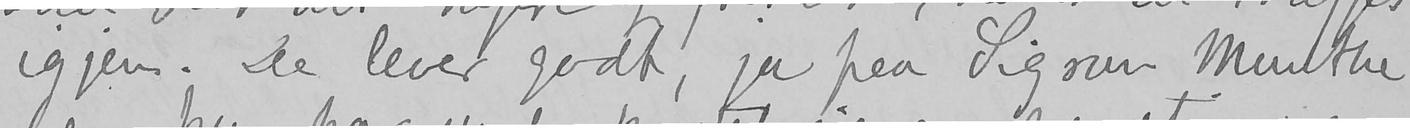igjen. De lever godt, ja paa Sigrun Munthe
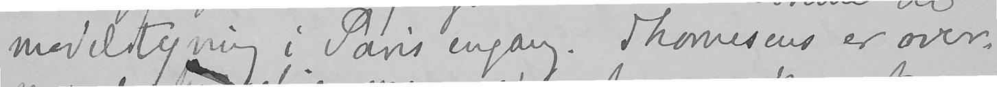modeltegning i Paris engang. Thomesens er over-
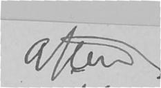aften
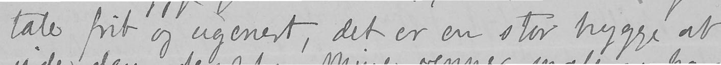tale frit og ugenert, det er en stor hygge at
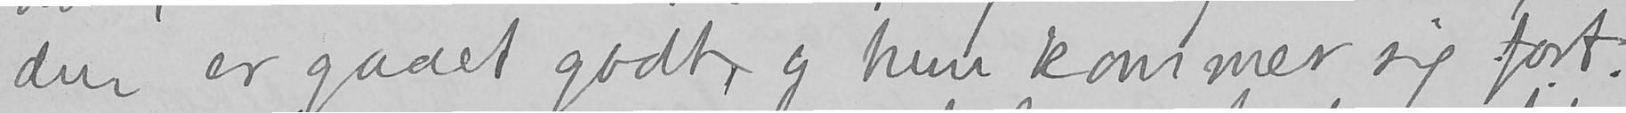den er gaaet godt, og hun kommer sig fort.
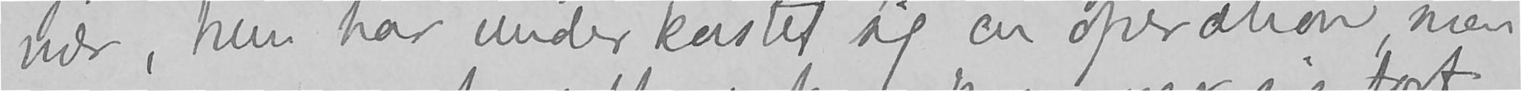nær, hun har underkastet sig en operation, men
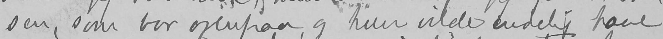sen, som bor ovenpaa, og hun vilde endelig have
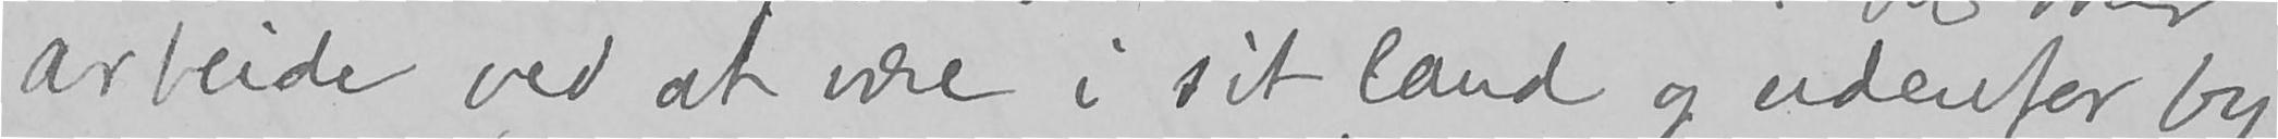arbeide ved at være i sit land og udenfor by,
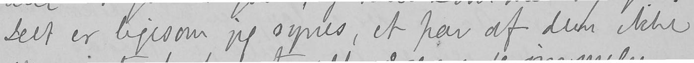Det er ligesom jeg synes, et par af dem ikke
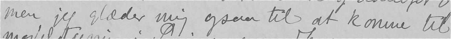men jeg glæder mig ogsaa til at komme til
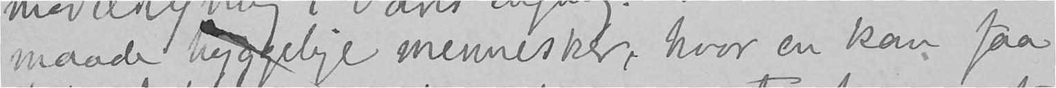maade hyggelige mennesker, hvor en kan faa
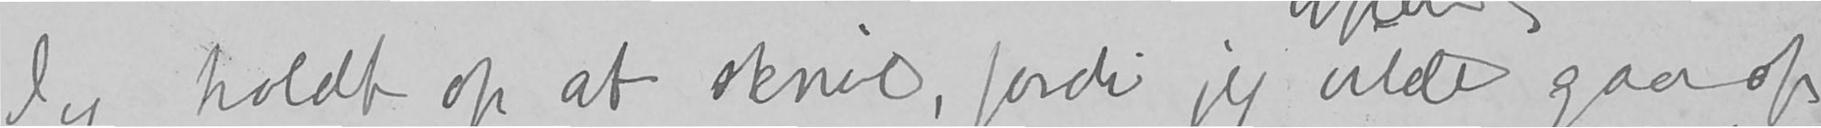Jeg holdt op at skrive, fordi jeg vilde gaa op
